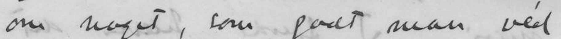om noget, som godt man vèd
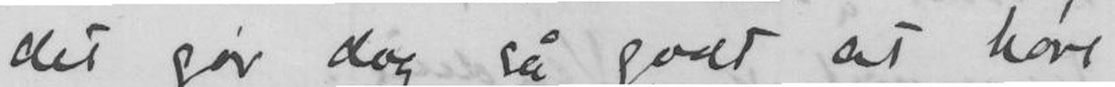det gør dog så godt at høre
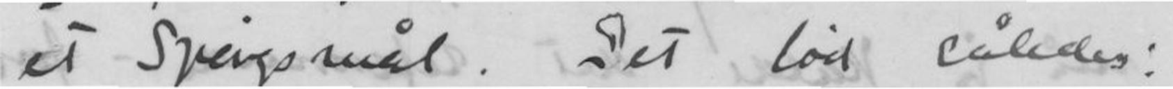et Spørgsmål. Det lød således:
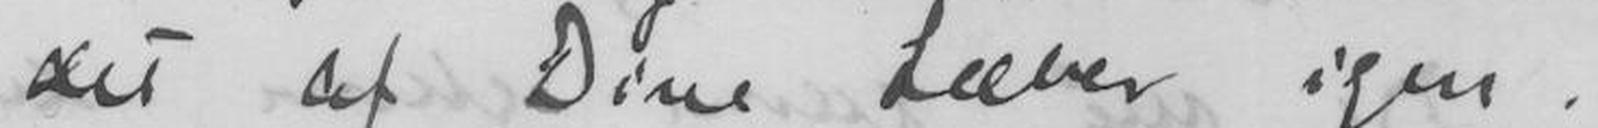det af dine Læber igen.
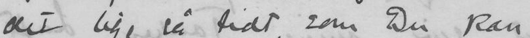det lige så tidt som Du kan,
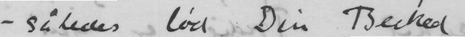- således lød Din Besked -
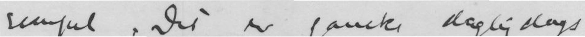sempel, Det er ganske dagligdags
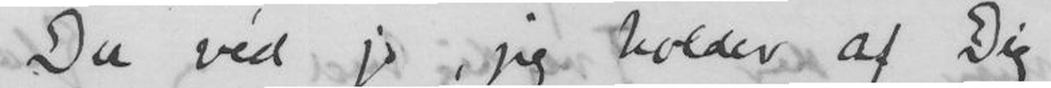Du vèd jo jeg holder af Dig
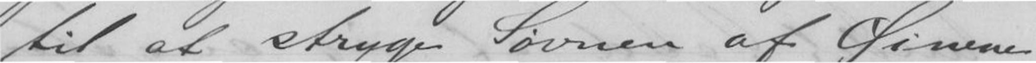til at stryge Søvnen af Øinene
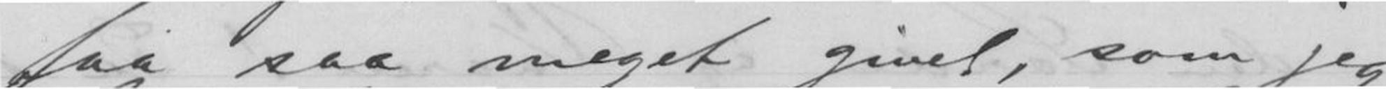faa saa meget givet, som jeg
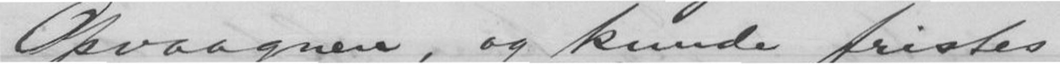Opvaagnen, og kunde fristes
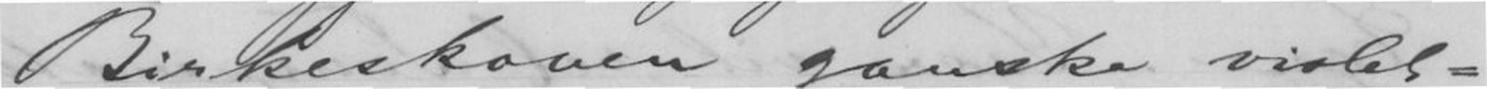Birkeskoven ganske violet-
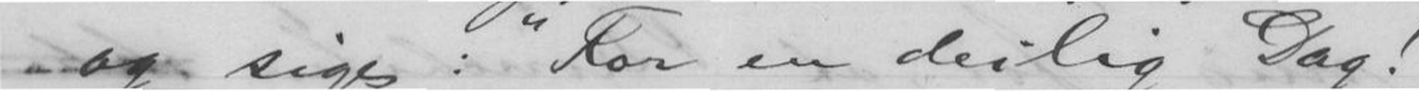og sige: "For en deilig Dag!"
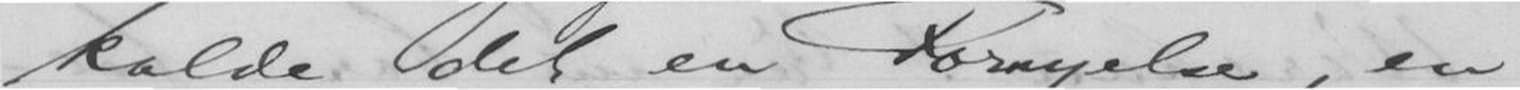kalde det en Fornyelse, en
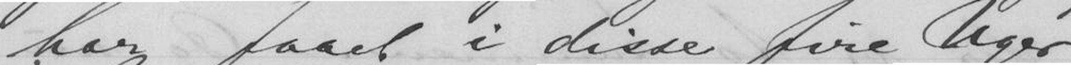har faaet i disse fire Uger
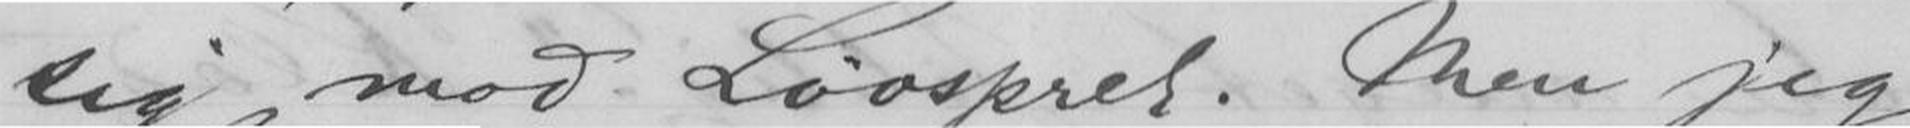sig mod Løvspret. Men jeg
